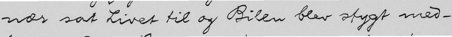nær sat Livet til og Bilen blev stygt med-
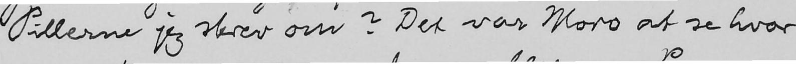Pillerne jeg skrev om? Det var Moro at se hvor
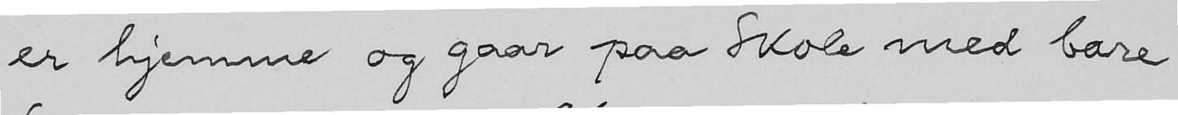er hjemme og gaar paa Skole med bare
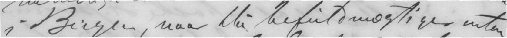i Bergen, naar Du befuldmægtiges enten
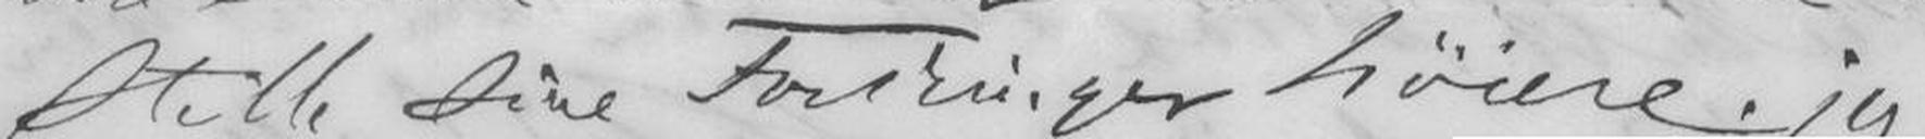Stille sine Fordringer høiere; jeg
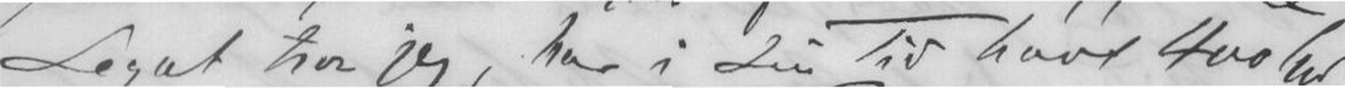Legat tror jeg, har i sin Tid havt 400 Spd,
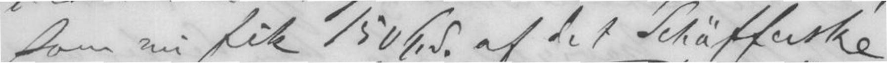som nu fik 150 Spd. af det Schäfferske
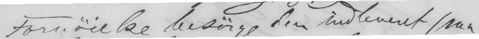Fornøilse besørge den indleveret paa
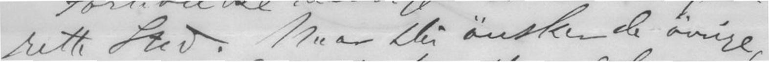rette Sted. Naar Du ønsker de øvrige
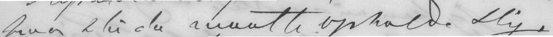hvor Du da maatte opholde Dig;
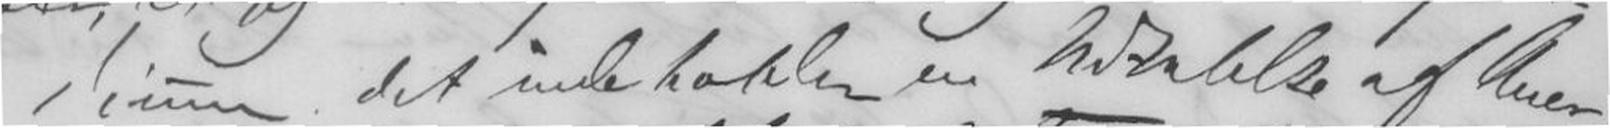dium; det indeholder en Udtalelse af Aner
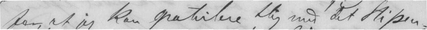for, at jeg kan gratulere Dig med det Stipen-
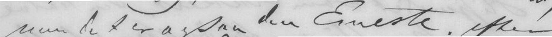men det er ogsaa den Eneste; efter
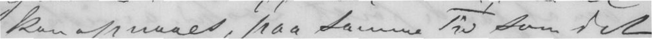kan opnaaes, paa samme Tid som det
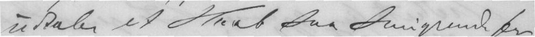udtaler et Haab saa smigrende for
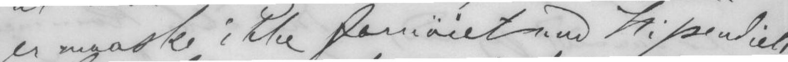er maaske ikke fornøiet med Stipendiets
